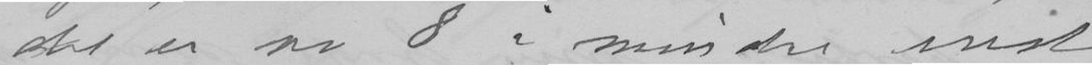det er nr 8 i mindre end
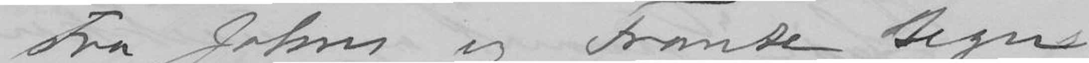Fra John og Frantz Beyer
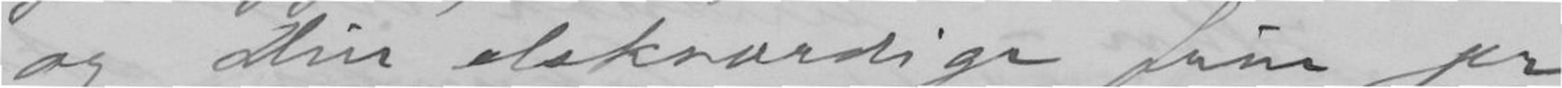og din elskværdige frue ser
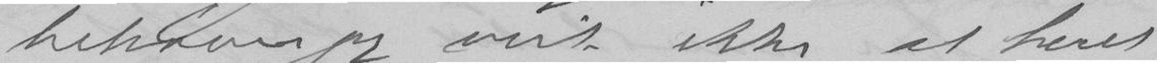behøver jeg vist ikke at beret-
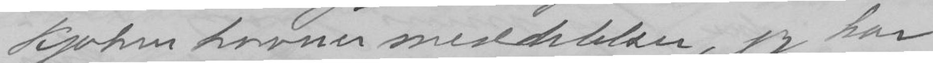Kjøbenhavner meddelelser, jeg har
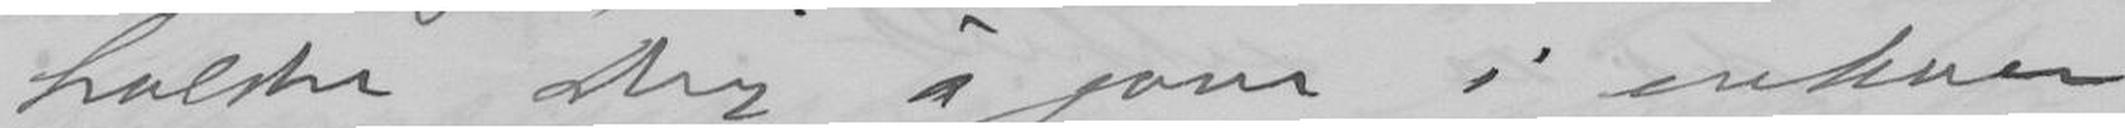holder Dig à jour i enhver
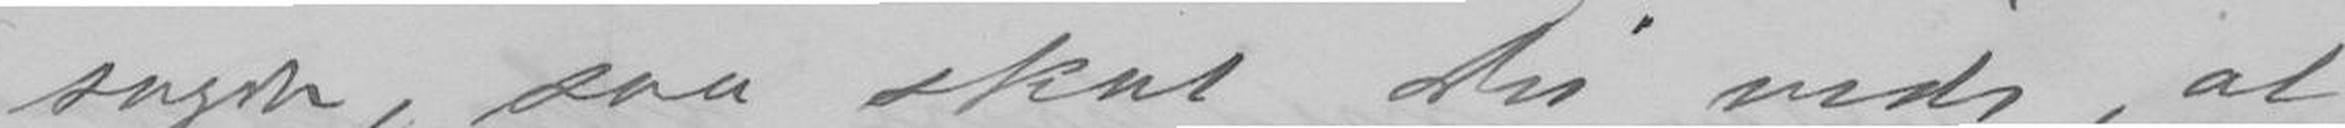sagte, saa skal Du vide, at
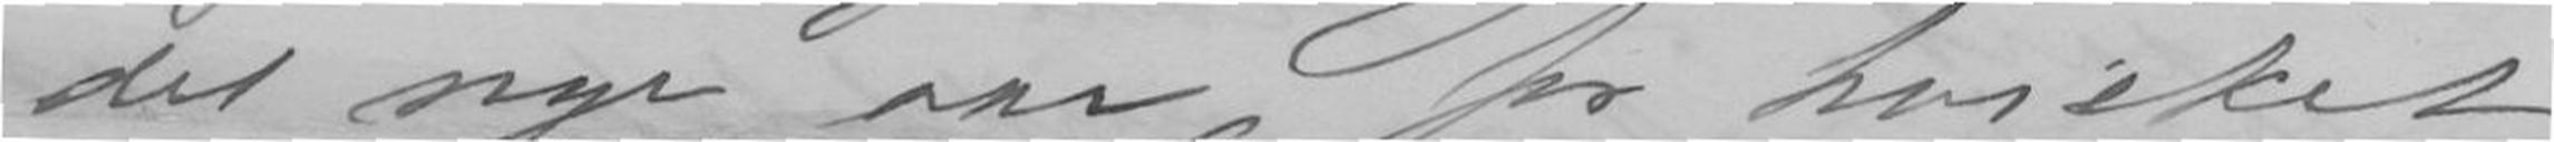det nye aar, for hvilket
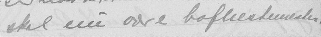skal nu være hofhestemester.
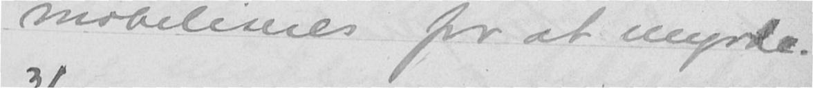mobiliseres for at myrde.
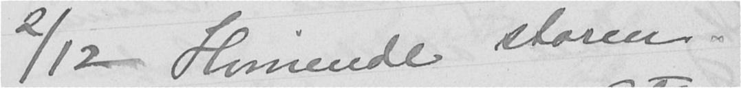2/12 Hvinende storm.
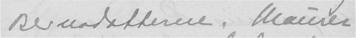Bernadotterne. Maurer
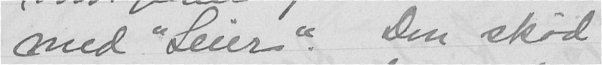med "Seier". Don skød
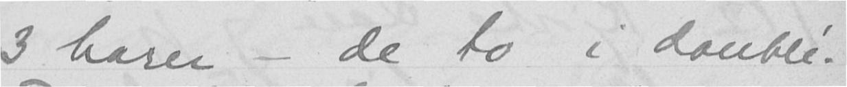3 harer - de to i double!
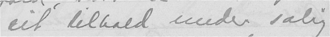sit tilhold under salig
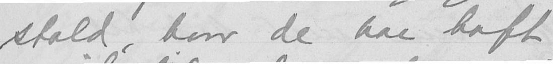stald, hvor de har haft
